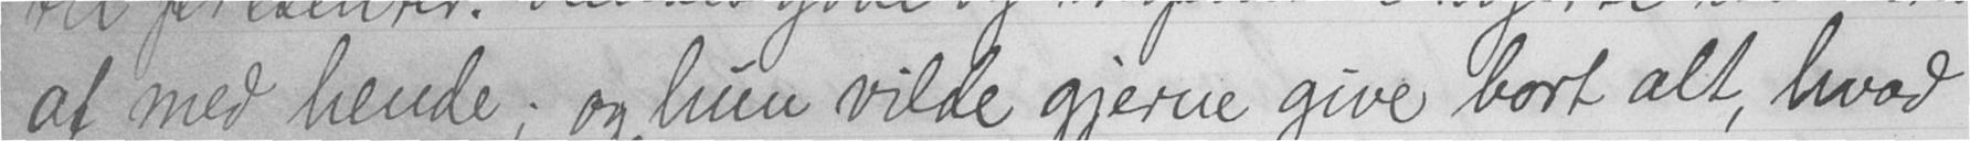af med hende; og hun vilde gjerne give bort alt, hvad
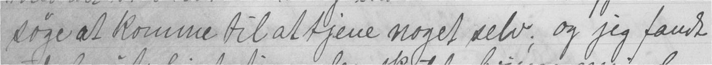søge at komme til at tjene noget selv; og jeg fandt
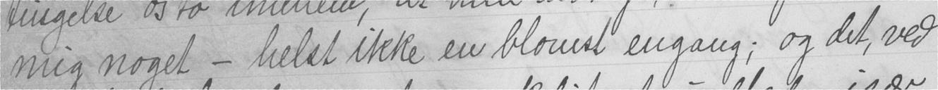mig noget - helst ikke en blomst engang; og det, ved
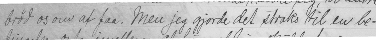brød os om at faa. Men jeg gjorde det straks til en be-
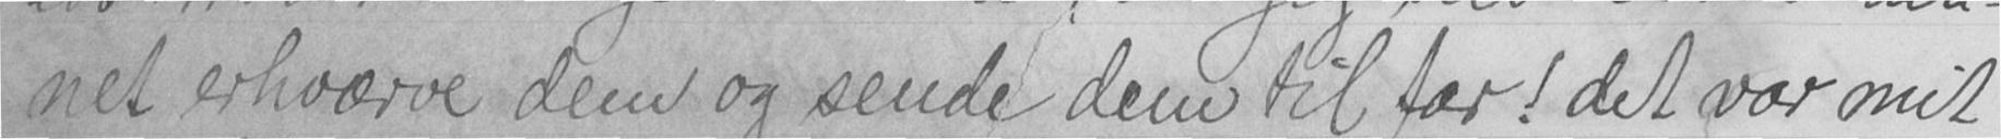net erhværve dem og sende dem til far! det var mit
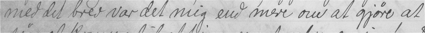med det brev var det mig end mere om at gjøre at
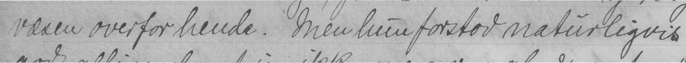væsen overfor hende. Men hun forstod naturligvis
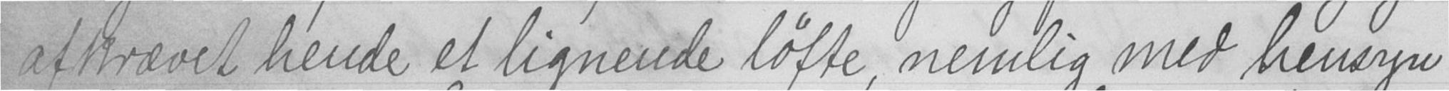afkrævet hende et lignende løfte, nemlig med hensyn
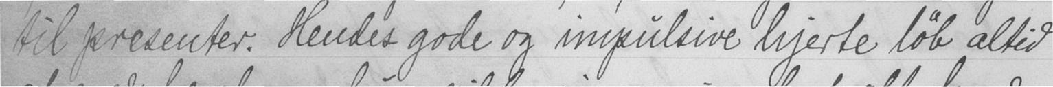til presenter. Hendes gode og impulsive hjerte løb altid
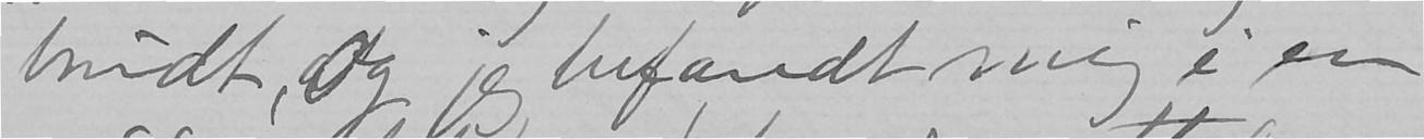brudt, og jeg befandt mig i en
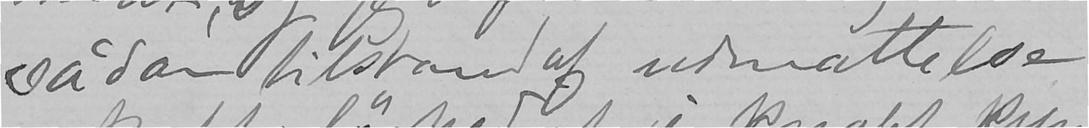sådan tilstand af udmattelse
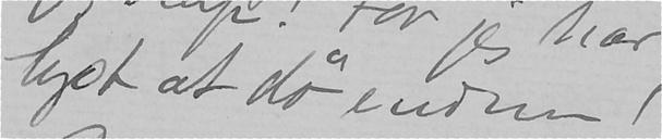lyst at dø endnu!
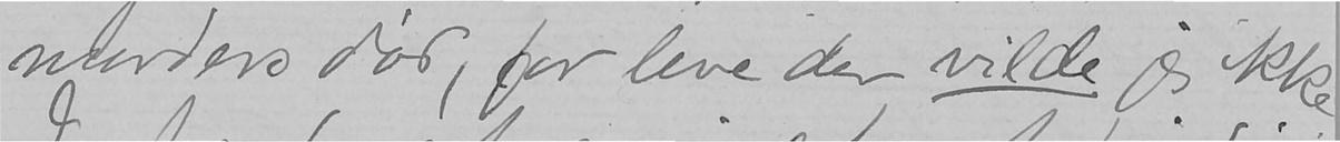morders død, for leve der vilde jeg ikke.
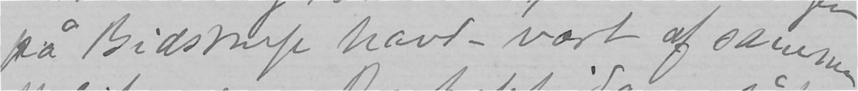på Bidstrup havde vært af samme
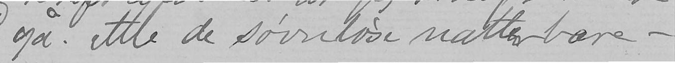gå. Alle de søvnløse nætter bare -
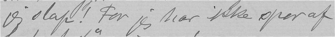jeg slap! For jeg har ikke spor af
